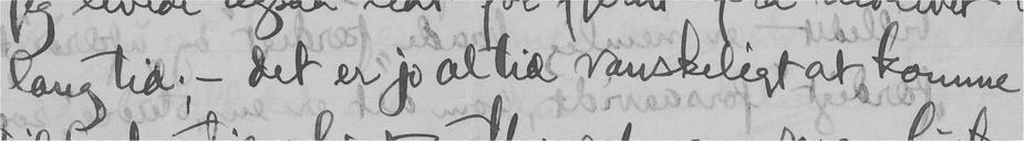lang tid; - det er jo altid vanskeligt at "komme
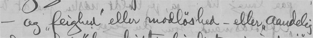- og "feighed" eller modløshed - eller "aandelig
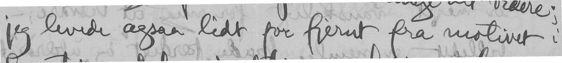jeg levede ogsaa lidt for fjernt fra motivet i
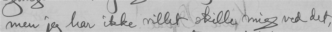men jeg har ikke villet skille mig ved det,
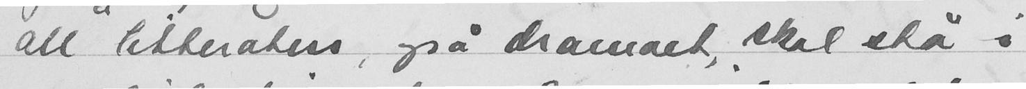all litteratur, også dramaet, skal stå i
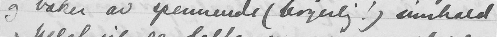og bøker av spennende (borgerlig!) innhold
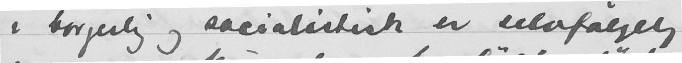i borgerlig og socialistisk er selvfølgelig
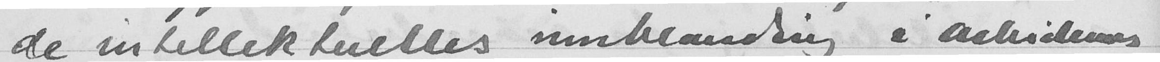de intellektuelles innblanding i arbidernes
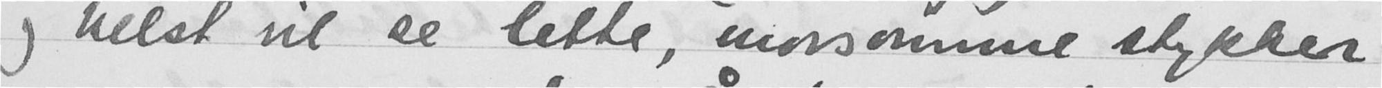og helst vil se lette, morsomme stykker
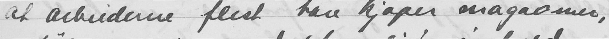at arbeiderne flest bare kjøper magasiner,
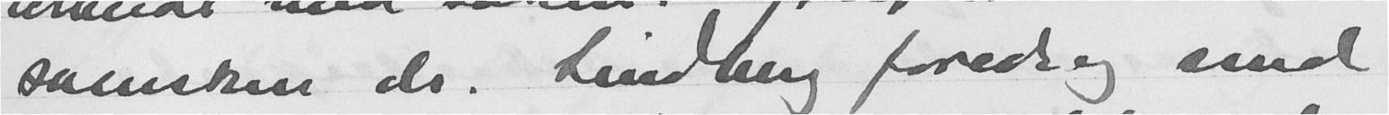svensken dr. Sindberg foredrag med
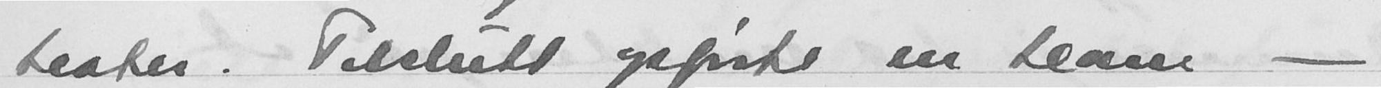teater. Tilslutt opførte en teane -
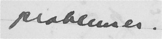problemer.
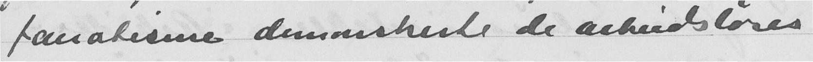fanatisme demonstrerte de arbeidsløses
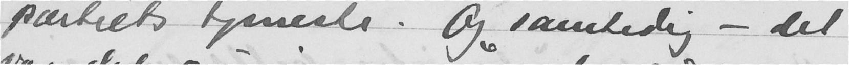partiets tjeneste. Og samtidig - det
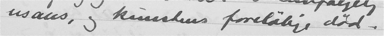usans, og kunstens foreløbige død.
